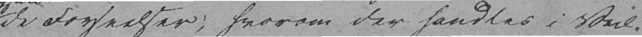de Forseelser, hvorom der handles i Veil.
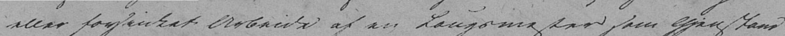eller forsinket Arbeide af en Laugsmester som Gjenstand
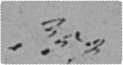353
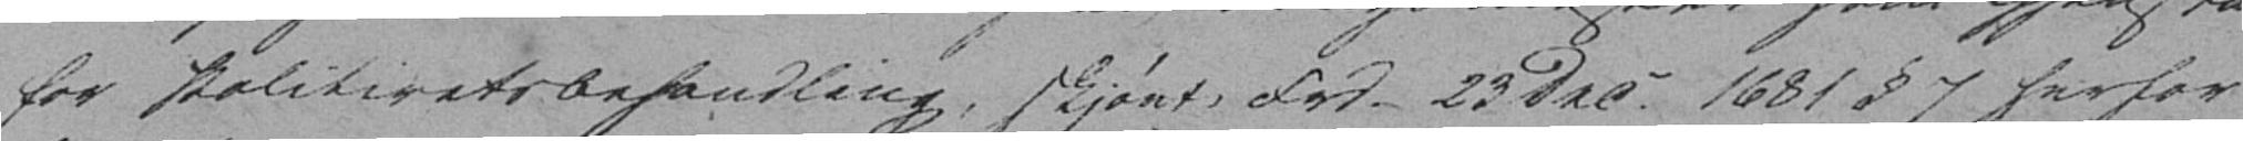for Politiretsbehandling, skjønt, Frd. 23 Dec. 1681 § 7 herfor
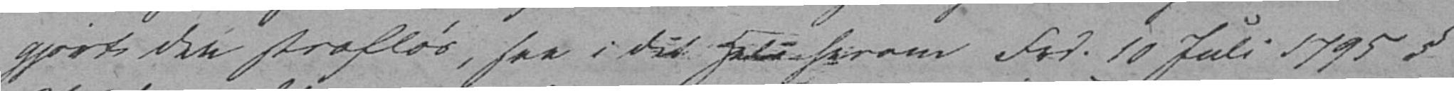gjort den strafløs, see i det Hele herom Frd. 10 Juli 1795 §
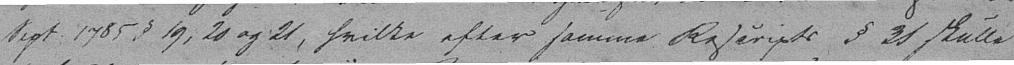Sept. 1785 § 19, 20 og 21, hvilke efter samme Rescripts § 31 skulle
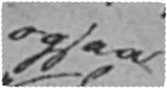ogsaa
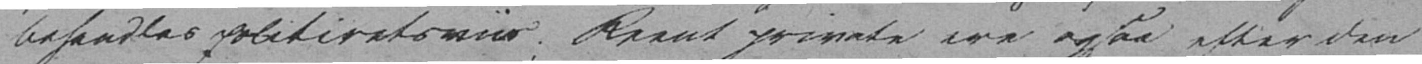behandles politiretsviis. Reent private ere ogsaa efter den
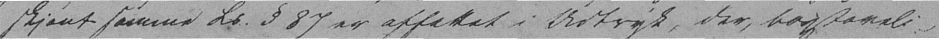skjønt samme Ls. § 87 er affattet i Udtryk, der, bogstaveli-
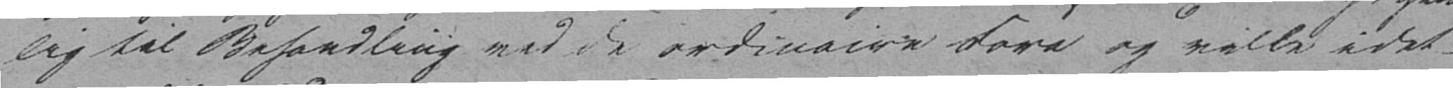lig til Behandling ved de ordnaire Fora og ville idet-
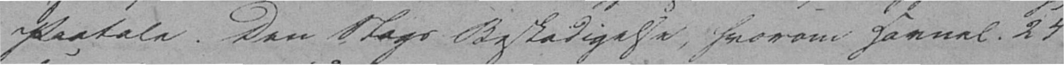Paatale. Den Slags Beskadigelse, hvorom Havnel. 24
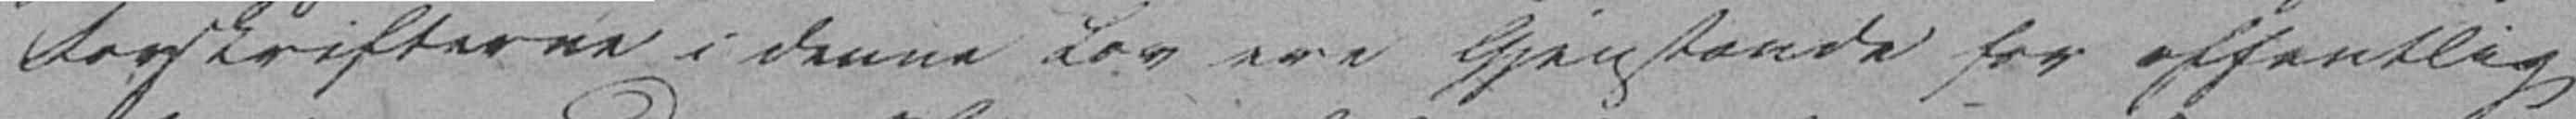Forskrifterne i denne Lov ere Gjenstande for offentlig
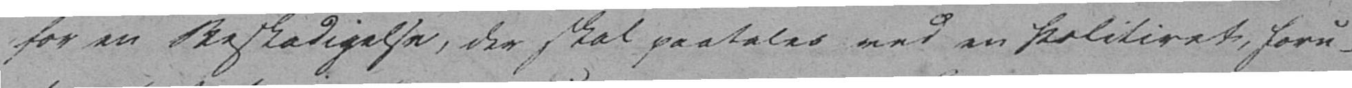for en Beskadigelse, der skal paatales ved en Politiret, foru-
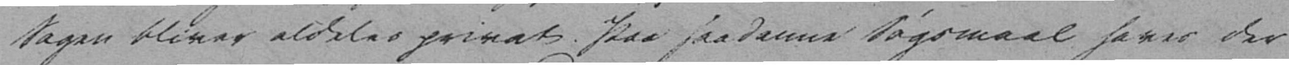Sagen bliver aldeles privat. Paa saadanne Søgsmaal haves der
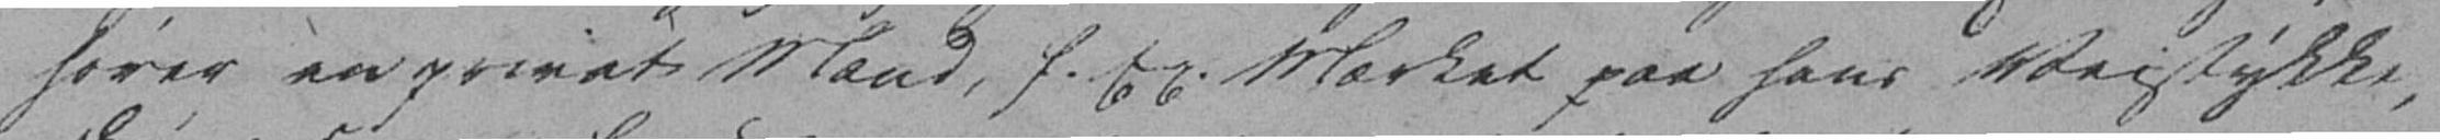hører en privat Mand, f. Ex. Mærket paa hans Veistykke,
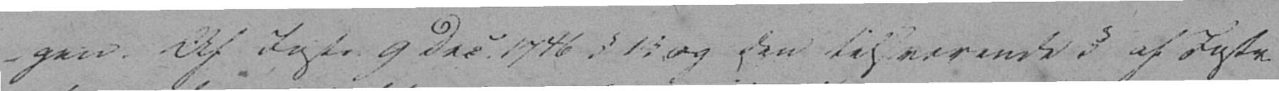gen. Af Instr. 9 Dec. 1746 § 13 og den tilsvarende § af Instr.
